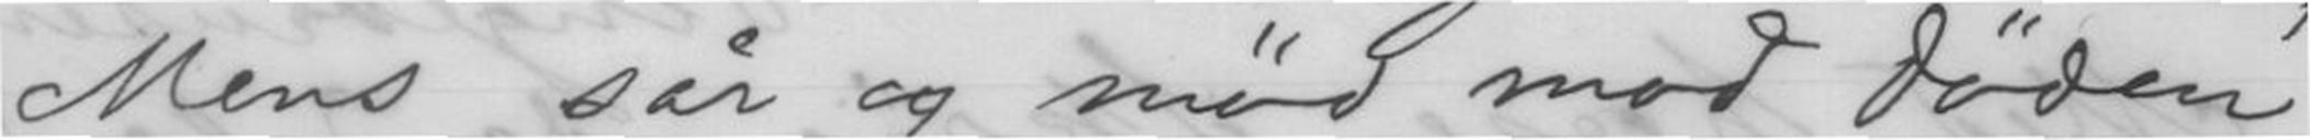Mens sår og mød mod døden
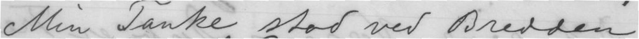Min Tanke stod ved Bredden
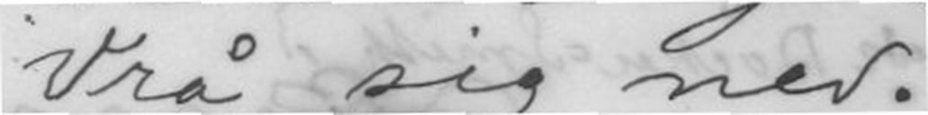Vrå sig ned.
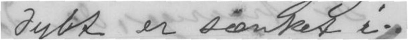dybt er sænket i.
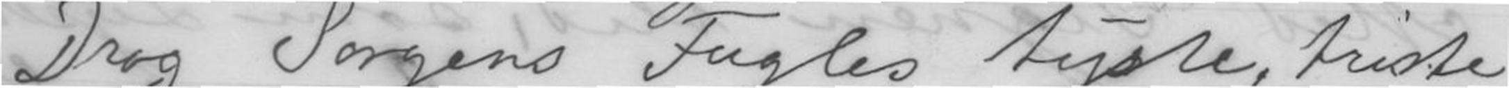Drog Sorgens Fugles tyste, triste
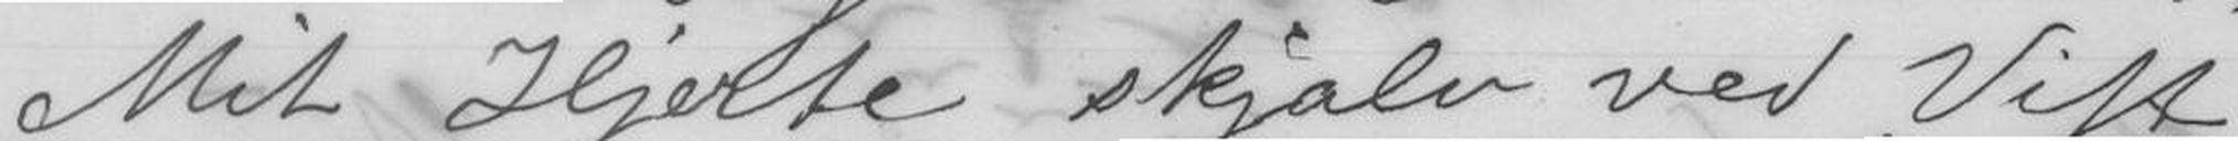Mit Hjerte skjalv ved Vift
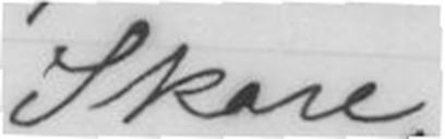Skare.
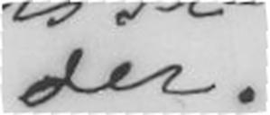der.
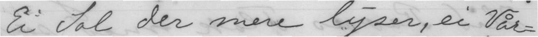Ei Sol der mere lyser, ei Vår-
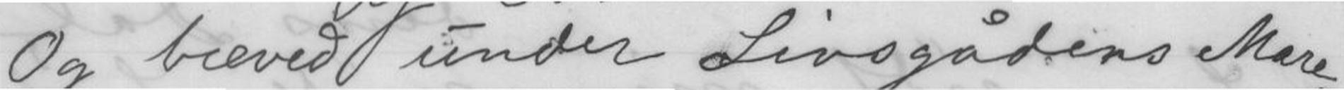Og bæred under Livsgårdens Mare.
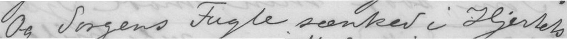Og Sorgens Fugle sænket i Hjertets
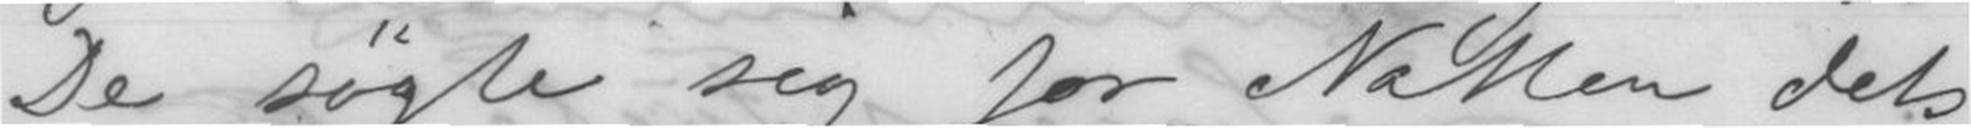De søgte sig for Natten dets
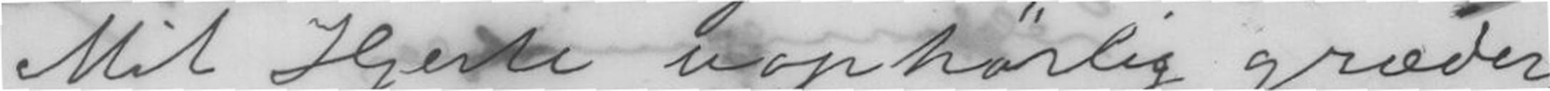Mit Hjerte uophørlig græder,
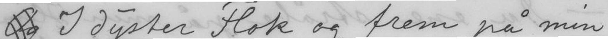Og I dyster og frem på min
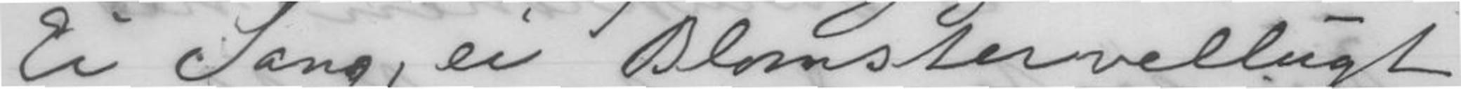Ei Sang, ei Blomstervellugt
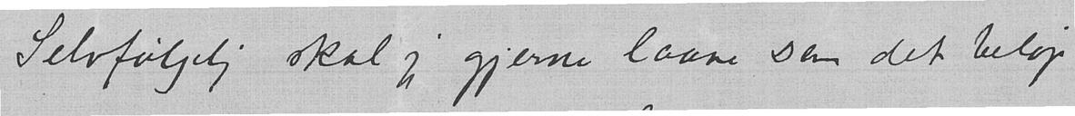Selvfølgelig skal jeg gjerne laane Dem det beløp
